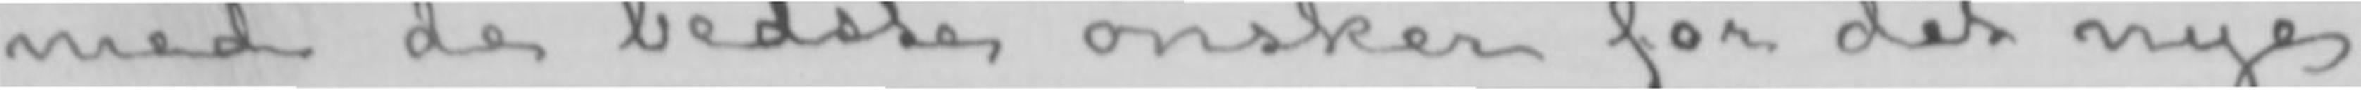med de bedste ønsker for det nye
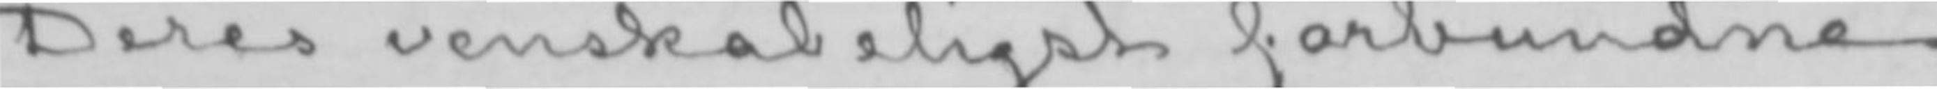Deres venskabeligst forbundne
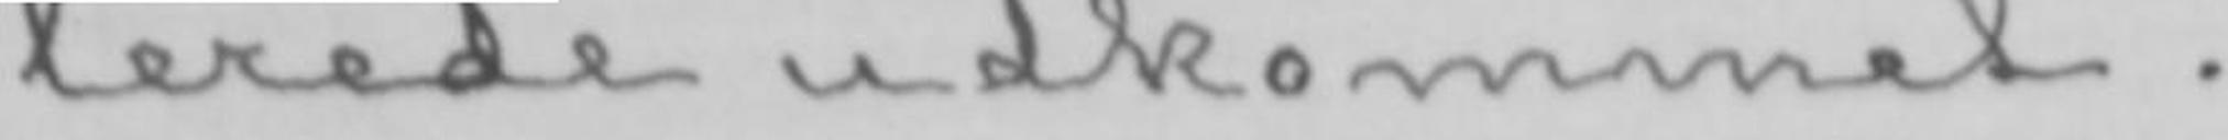lerede udkommet.
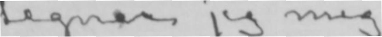tegner jeg mig
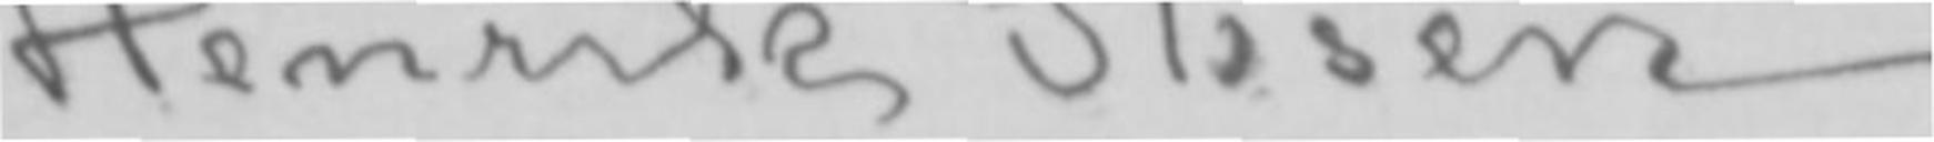Henrik Ibsen.
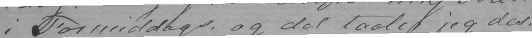i Formiddag, og det taaler jeg des-
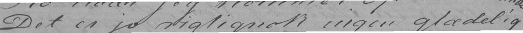Det er jo rigtignok ingen glædelig
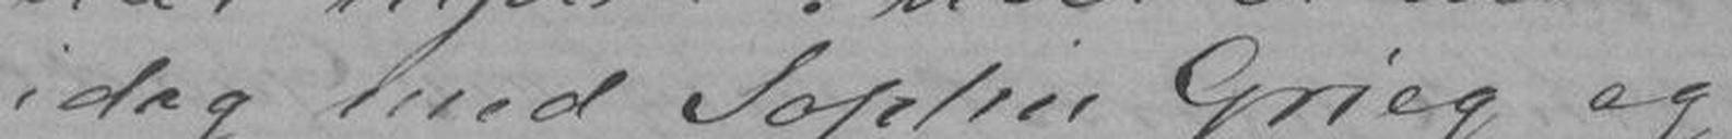idag med Sophie Grieg og
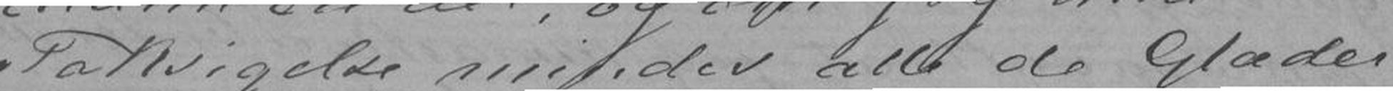Taksigelse mindes alle de Glæder
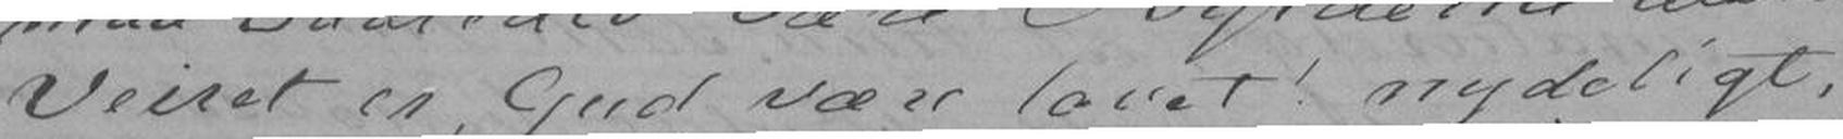Veiret er, Gud være lovet! nydeligt,
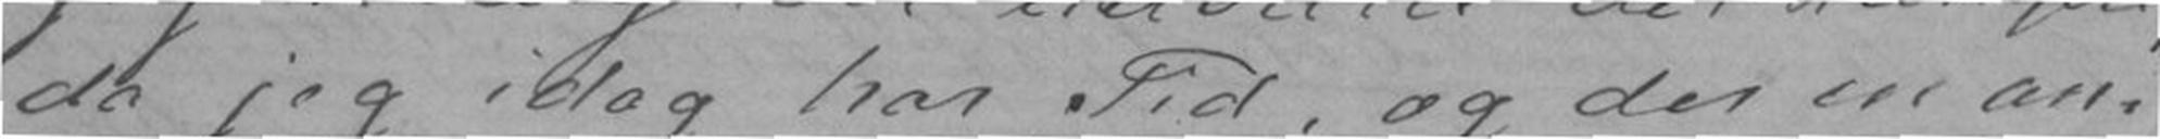da jeg idag har Tid, og der en an-
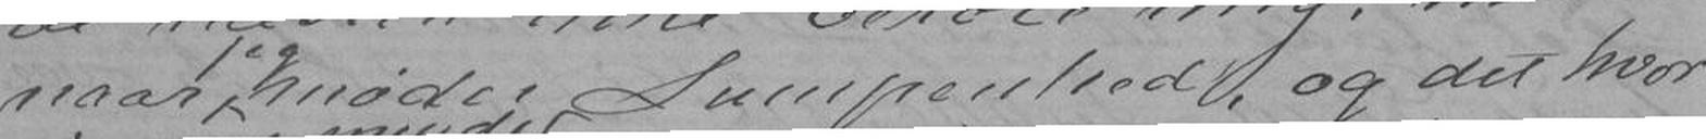naar jeg møder Lumpenhed, og det hvor
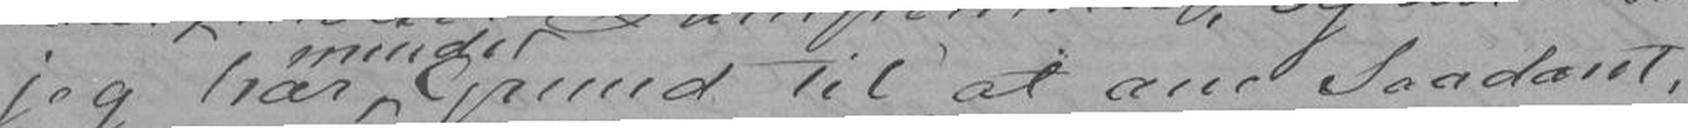jeg har mindst Grund til at ane Saadant,
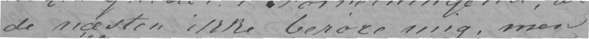de næsten ikke berøre mig, men
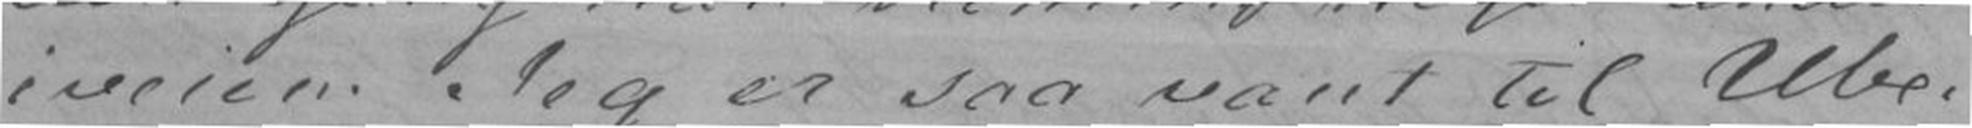iveien. Jeg er saa vant til Ube-
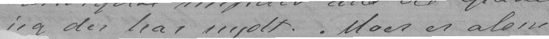jeg der har nydt. Moer er alene
